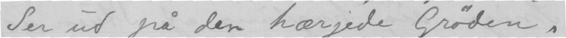Ser ud på den hærjede Grøden.
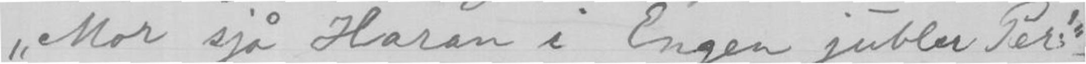"Mor sjå Haran i Engen jubler Per!"
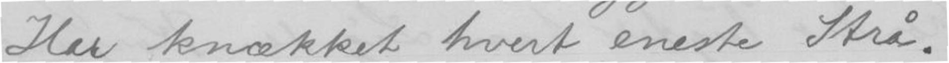Her knækket hver eneste strå.
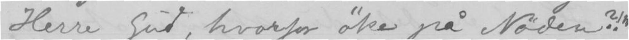Herre Gud, hvorfor øke på Nøden?!"
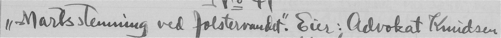"Martsstemning ved Jølstervandet". Eier: Advokat Knudsen
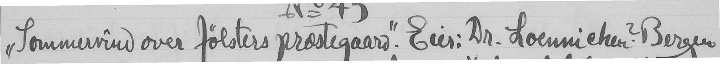"Sommervind over Jølsters præstegaard" Eier: Hr. Loennichen? Bergen
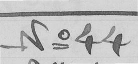No 44
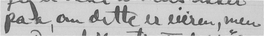pat, om dette er eieren, men
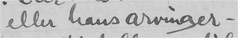eller hans arvinger -
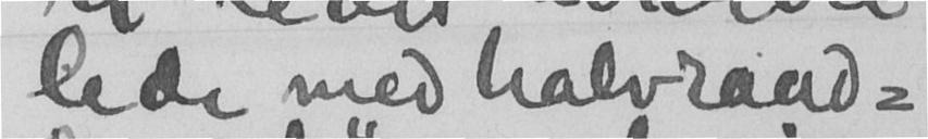lede med halvraud-
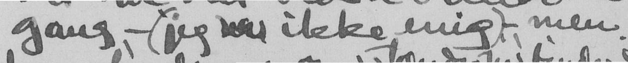gang - (jeg var ikke enig), men
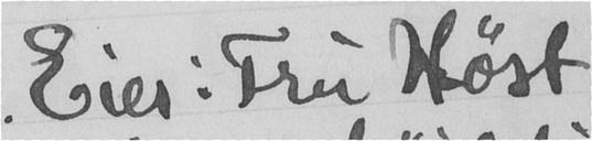Eier: Fru Høst
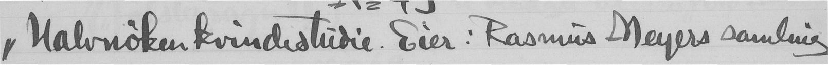"Halvnøken kvindestudie". Eier: Rasmus Meyers samling
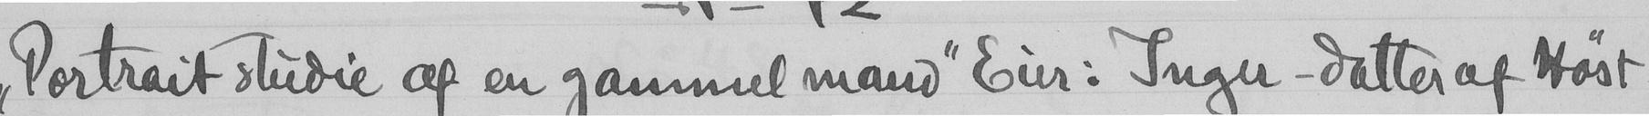"Portrait studie af en gammel mand" Eier: Inger - datter af Høst
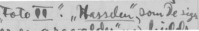"Foto II". "Hasselen", som De sign
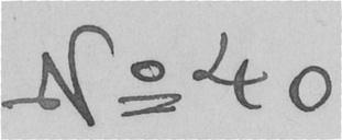No 40
